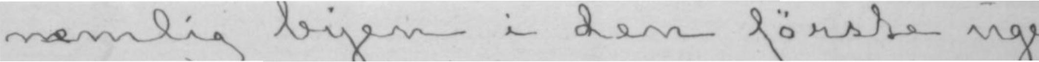nemlig byen i den første uge
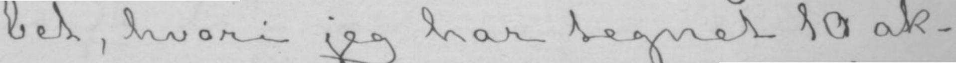bet, hvori jeg har tegnet 10 ak-
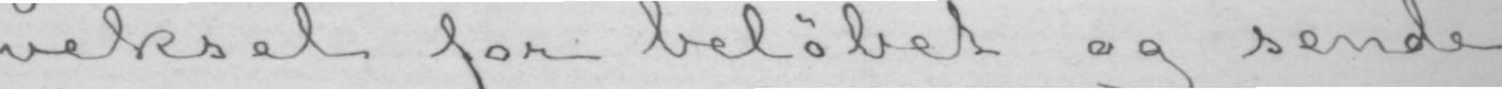veksel for beløbet og sende
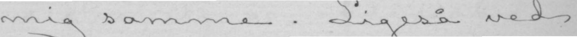mig samme. Ligeså ved
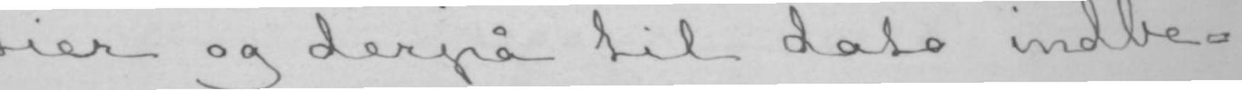tier og derpå til dato indbe-
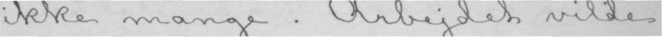ikke mange. Arbejdet vilde
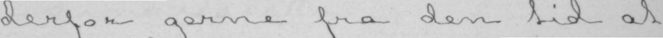derfor gerne fra den tid at
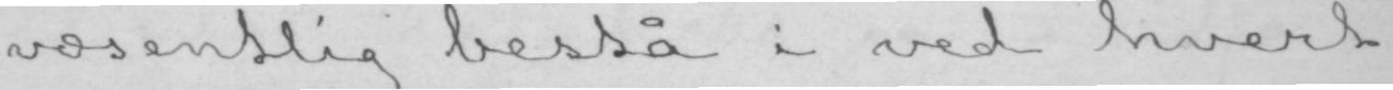væsentlig bestå i ved hvert
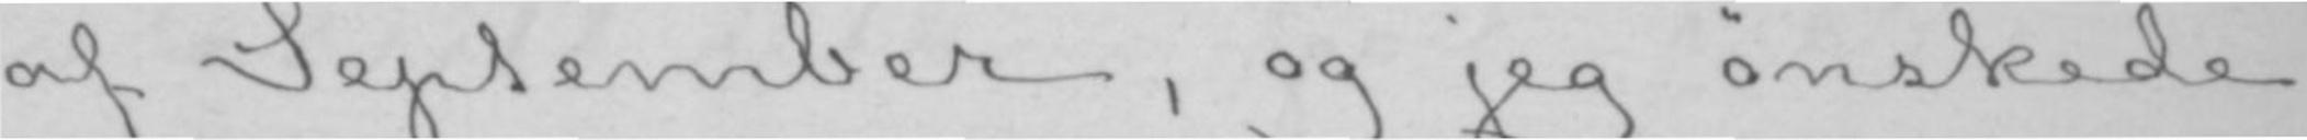af September, og jeg ønskede
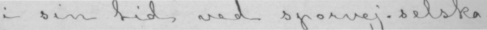i sin tid ved sporvej-selska-
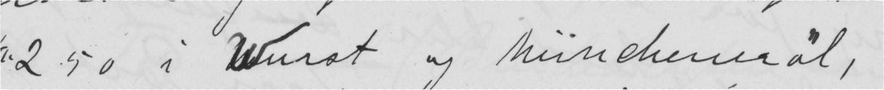2. 50 i Wurst og Munchenerøl,
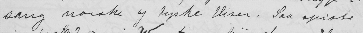sang norske og tyske Viser. Saa spiste
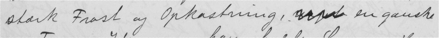stærk Frost og Opkastning,  en ganske
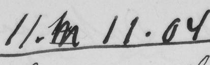11. 11.04
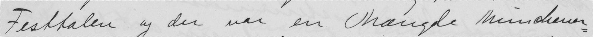Festtalen og der var en Mængde Münchener-
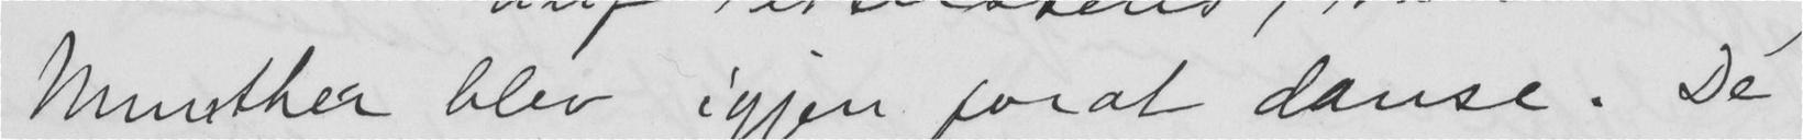Munther blev igjen forat danse. De
